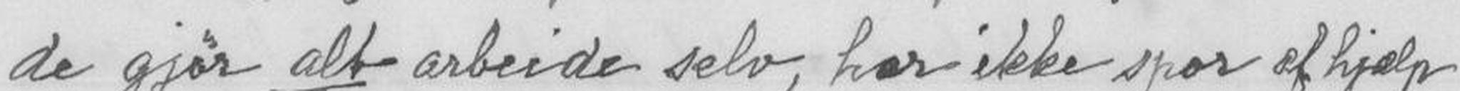de gjør alt arbeide selv, tar ikke spor af hjælp
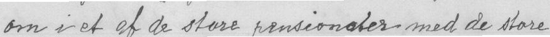om i et af de store pensionater med de store
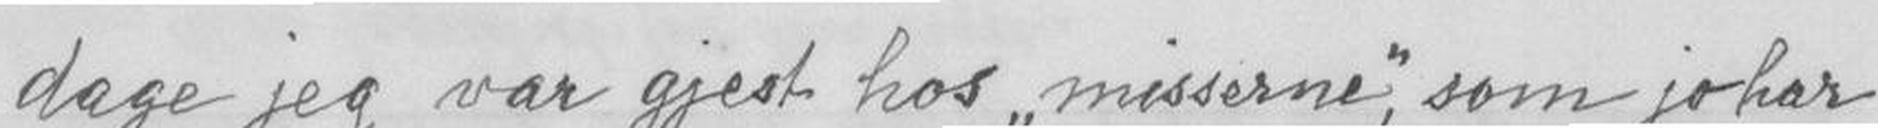dage jeg var gjest hos "misserne", som jo har
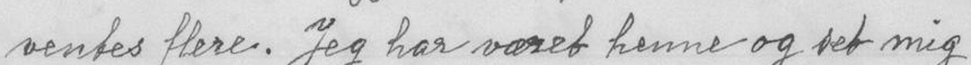ventes flere. Jeg har været hemme og set mig
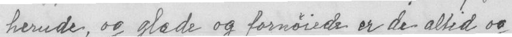herude, og glade og fornøiede er de altid og
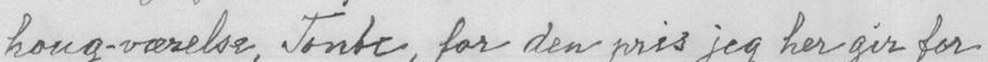haug-værelse, Tonte, for den pris jeg her gir for
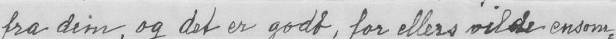fra dem, og det er godt, for ellers vilde ensom-
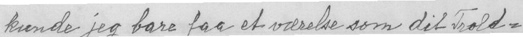kunde jeg bare faa et værelse som dit Trold-
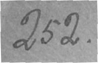252.
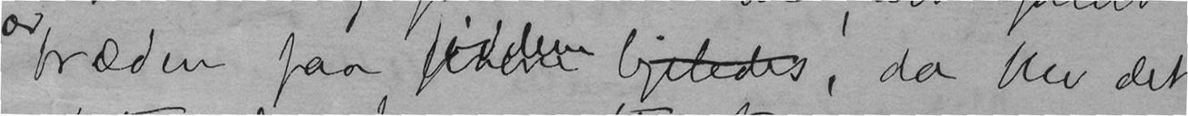træden paa fødderne ligeledes, da blev det
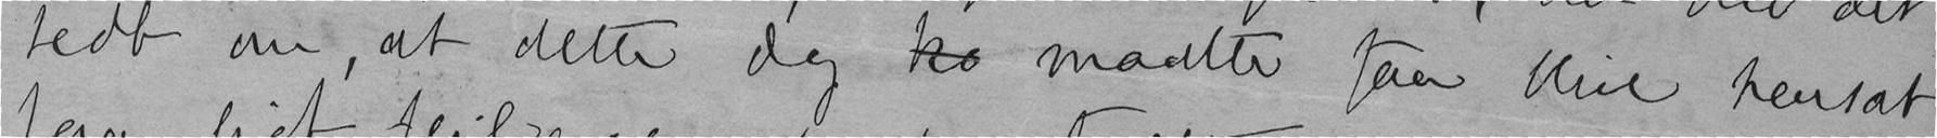bedt om, at dette dog ko maatte faa blive hensat
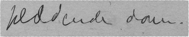fældende dom.
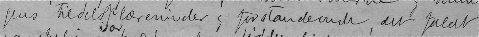gens tildels oplærerinder og forstandernde, der faldt
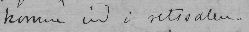komme ind i retssalen.
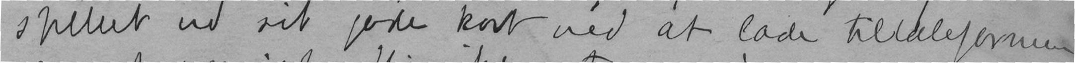spillet ud sit gode kort ved at lade tilaleformen
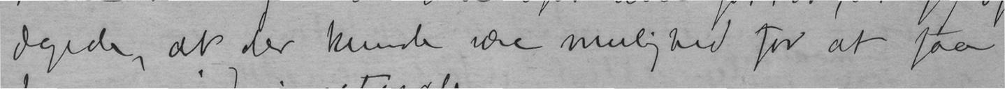dagede, at der kunde være mulighed for at faa
